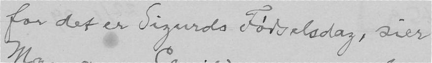for det er Sigurds Fødselsdag, sier
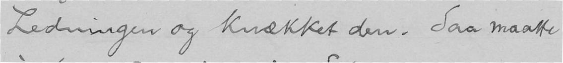Ledningen og knækket den. Saa maatte
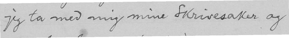jeg ta med mig mine Skrivesaker og
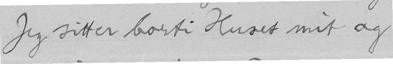Jeg sitter borti Huset mit og
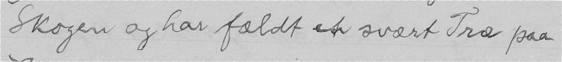Skogen og har fældt et svært Træ paa
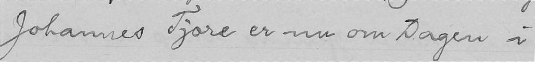Johannes Tjore er nu om Dagen i
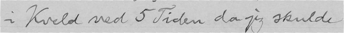i Kveld ved 5 Tiden da jeg skulde
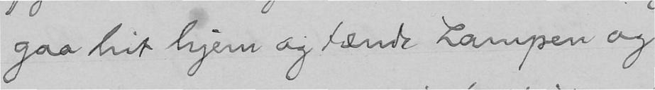gaa hit hjem og tænde Lampen og
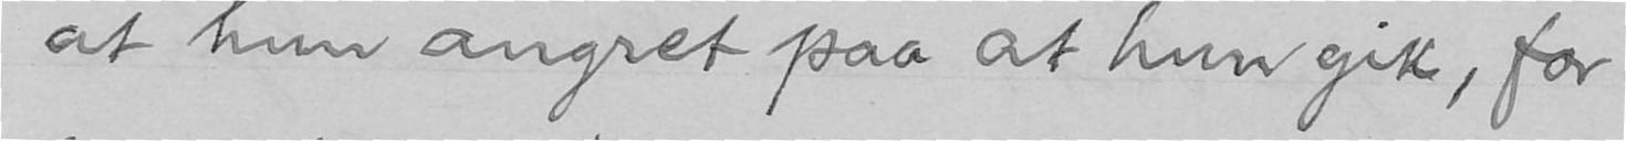at hun angret paa at hun gik, for
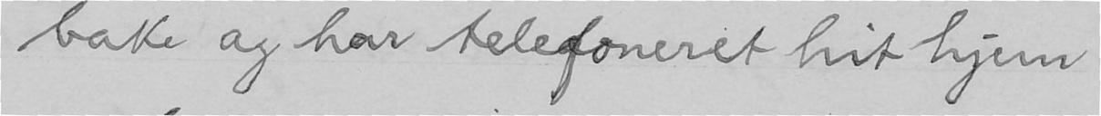bake og har telefoneret hit hjem
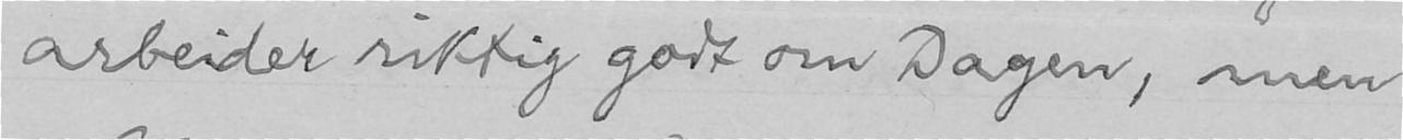arbeider riktig godt om Dagen, men
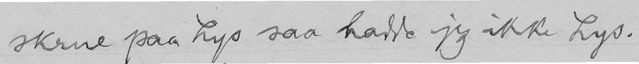skrue paa Lys saa hadde jeg ikke Lys.
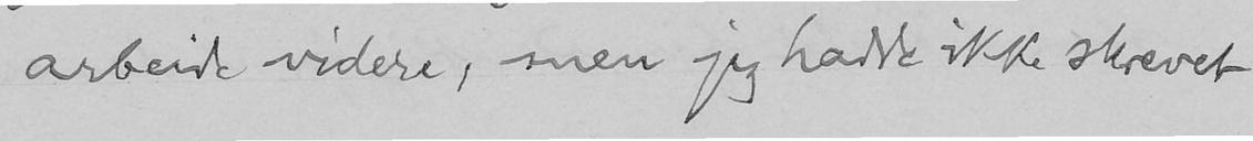arbeide videre, men jeg hadde ikke skrevet
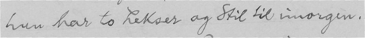hun har to Lekser og Stil til imorgen.
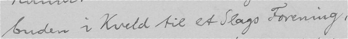buden i Kveld til et Slags Forening,
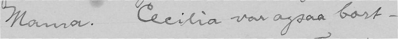Mama. Cecilia var ogsaa bort-
